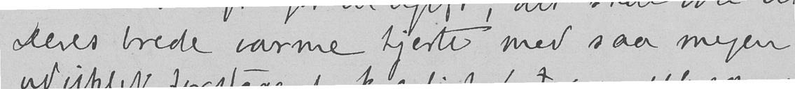Deres brede varme hjerte med saa megen
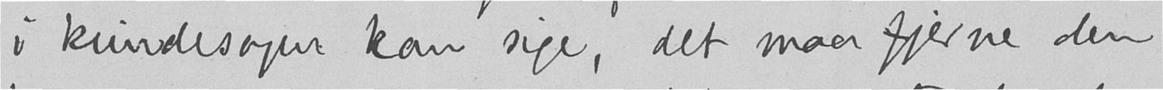i kvindesagen kan sige, det maa fjerne den
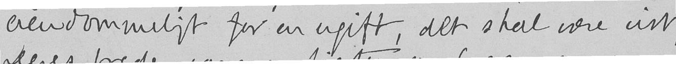eiendommeligt for en ugift, det skal være vist,
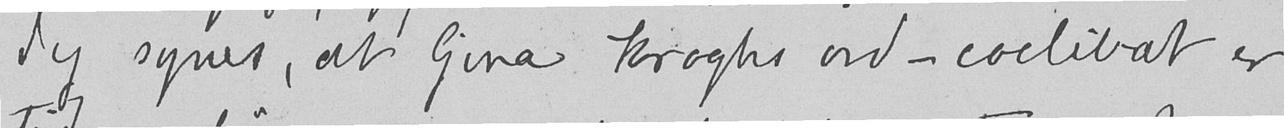Jeg synes, at Gina Kroghs ord - coelibat er
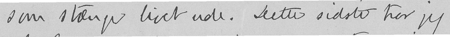som stænger livet ude. Dette sidste tror jeg
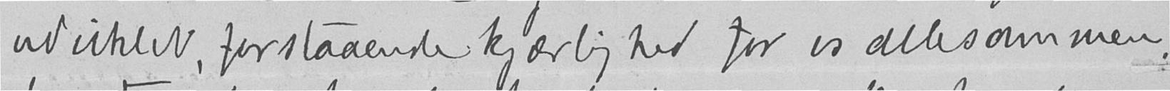uviklet, forstaaende kjærlighed for os allesammen.
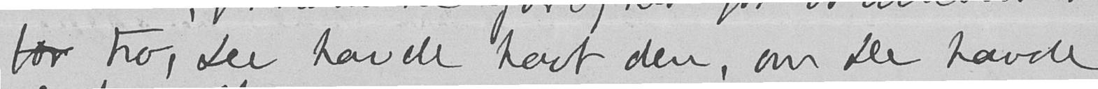bor tro, De havde havt den, om de havde
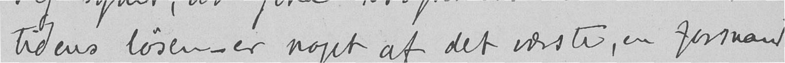tidens løsen - er noget af det værste, en formand
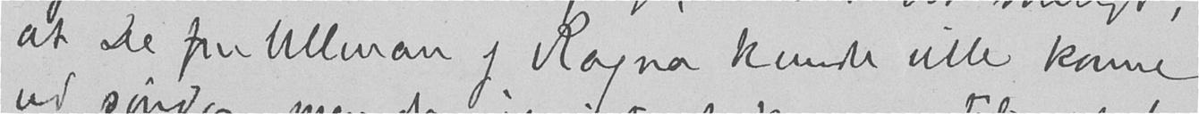at De fru Ullman og Ragna kunde ville komme
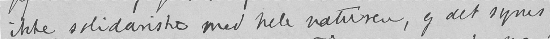ikke solidarisk med hele naturen, og det synes
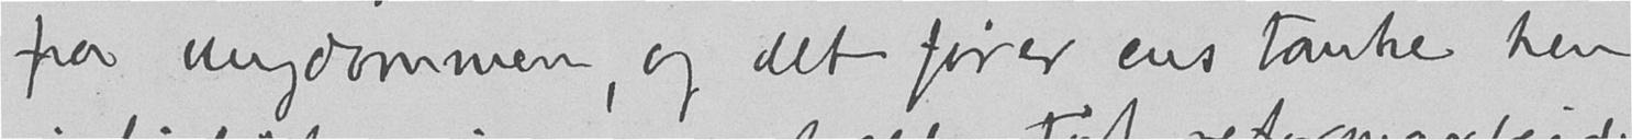fra ungdommen, og det fører ens tanke hen
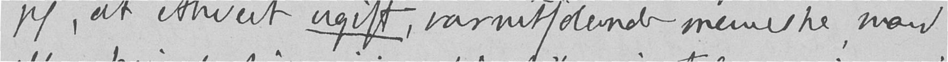jeg at ethvert ugift, varmtfølende menneske, mand
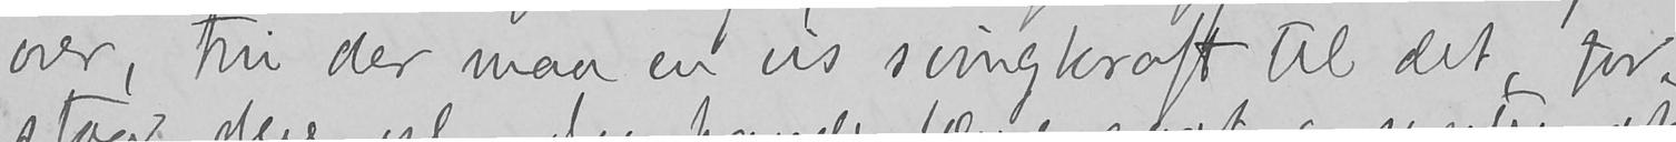over, thi der maa en vis svingkraft til det, for-
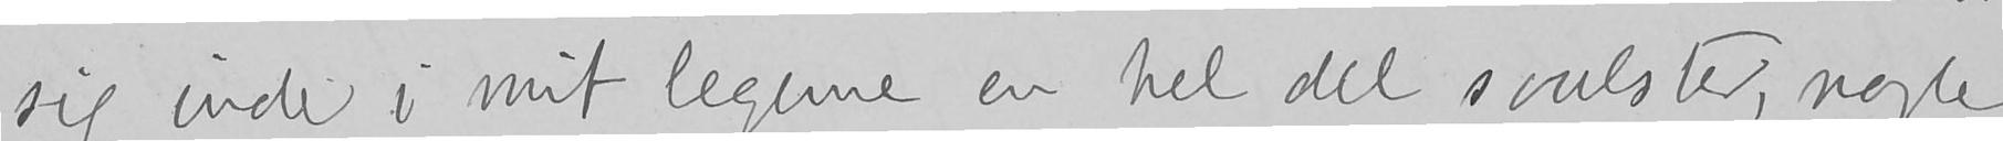sig inde i mit legeme en hel del svulster, nogle
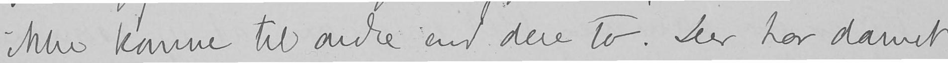ikke komme til andre end dere to. Der har dannet
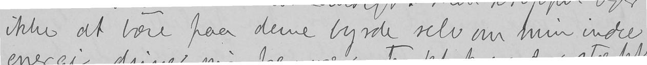ikke at bære paa denne byrde selv om min indre
